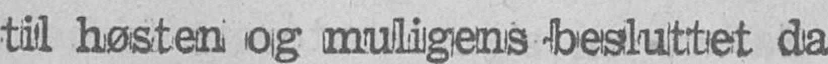til høsten og muligens besluttet da.
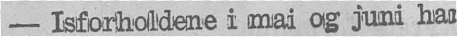- Isforholdene i mai og juni har
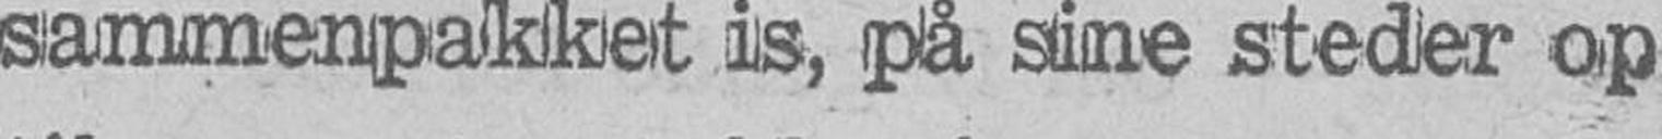sammenpakket is, på sine steder op-
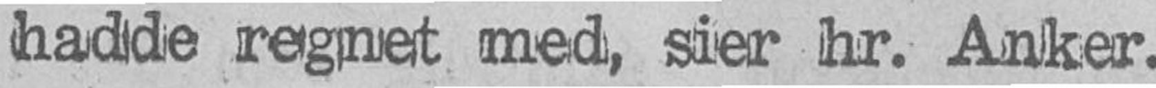hadde regnet med, sier hr. Anker.
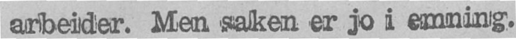arbeider. Men saken er jo i emning.
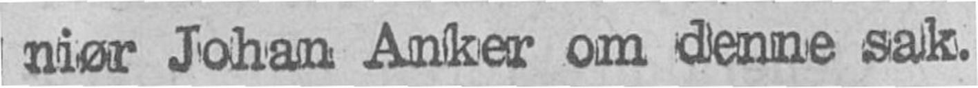niør Johan Anker om denne sak.
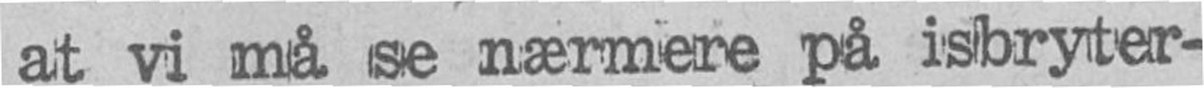at vi må se nærmere på isbryter-
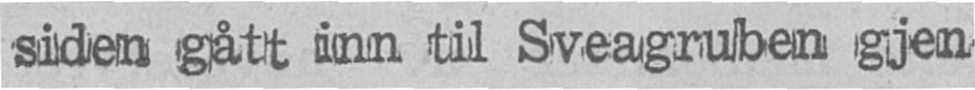siden gått inn til Sveagruben gjen-
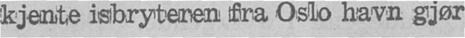kjente isbryteren fra Oslo havn gjør
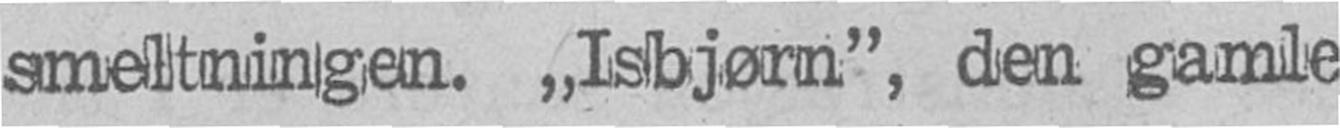smeltningen. „Isbjørn", den gamle
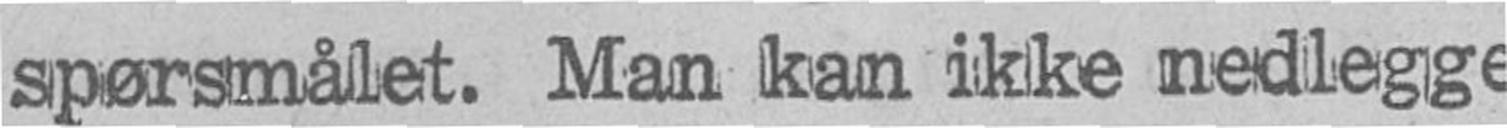spørsmålet. Man kan ikke nedlegge
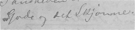Gode og det Skjønne.
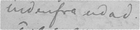indenfra udad.
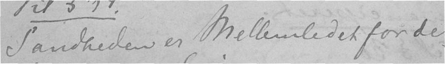Sandheden er Mellemledet for det
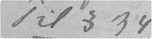Til § 34
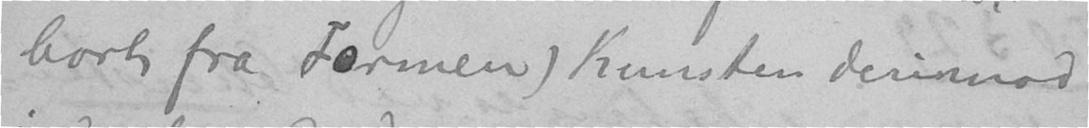bort fra Formen) Kunsten derimod
